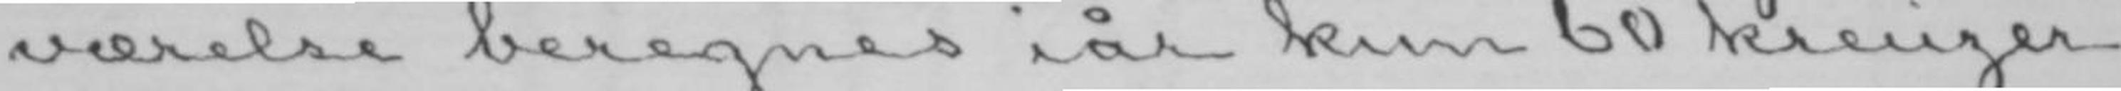værelse beregnes iår kun 60 kreuzer
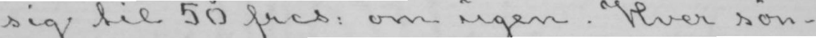sig til 50 frcs: om ugen. Hver søn-
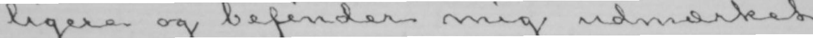ligere og befinder mig udmærket
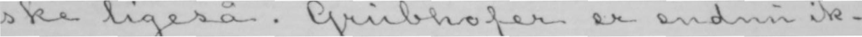ske ligeså. Grubhofer er endnu ik-
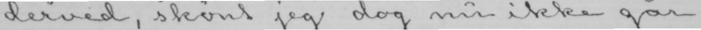derved, skønt jeg dog nu ikke går
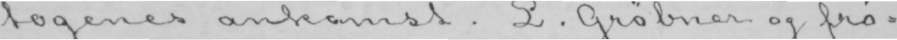togenes ankomst. L. Gröbner og frø-
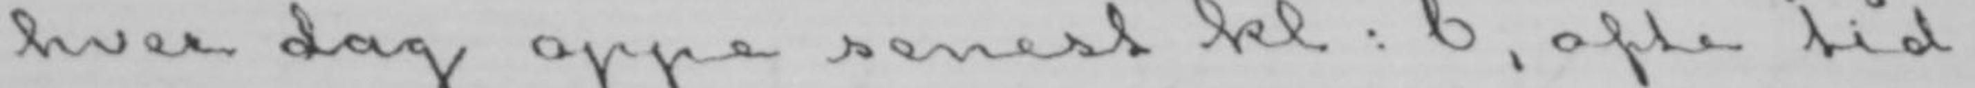hver dag oppe senest kl: 6, ofte tid-
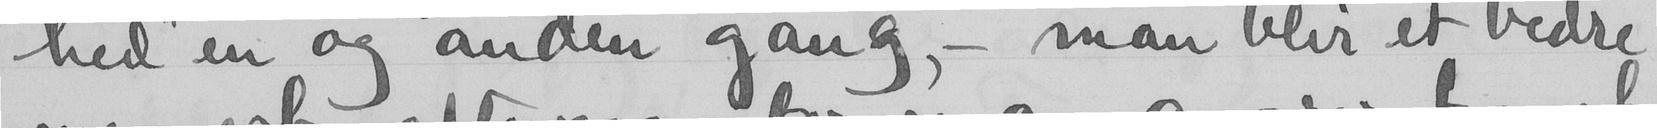hed" en og anden gang, - man blir et bedre
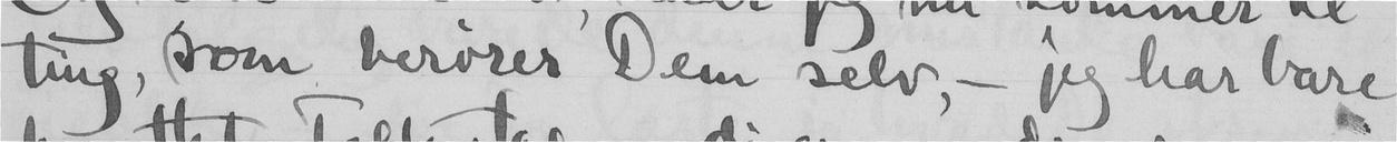ting, som berører Dem selv - jeg har bare
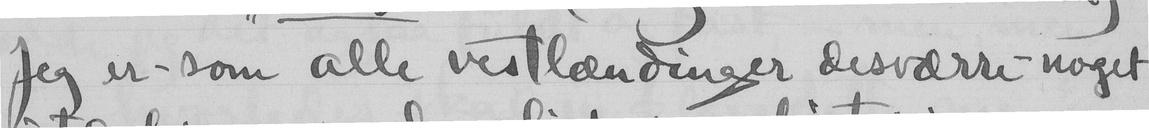Jeg er - som alle vestlændinger desværre - noget
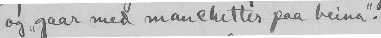og "gaar" med manchetter paa beina".
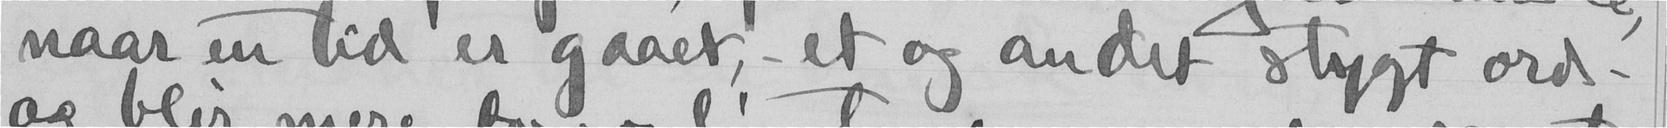naar en tid er gaaet, - et og andet stygt ord
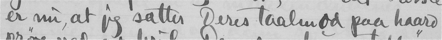er nu, at jeg sætter Deres taalmod paa haard
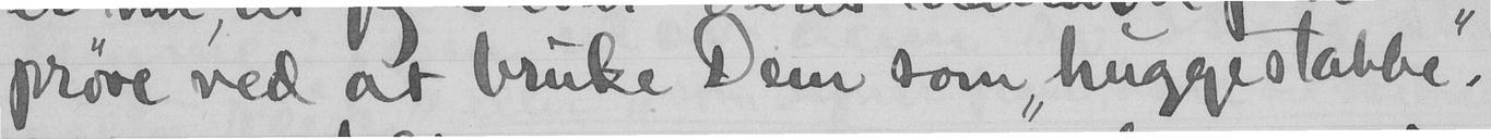prøve ved at bruke Dem som "huggestabbe".
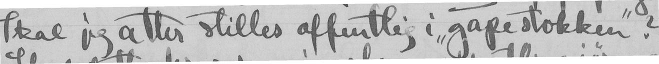Skal jeg atter stilles offentlig i "gapestokken"?
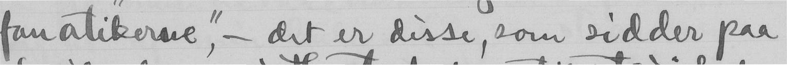fanatikerne", - det er disse, som sidder paa
